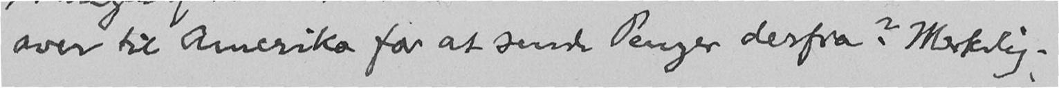over til Amerika for at sende Penger derfra? Merkelig.
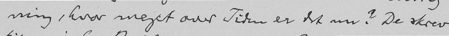ning, hvor meget over Tiden er det nu? De skrev
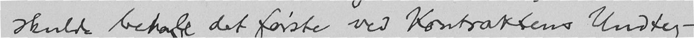skulde betale det første ved Kontraktens Undteg-
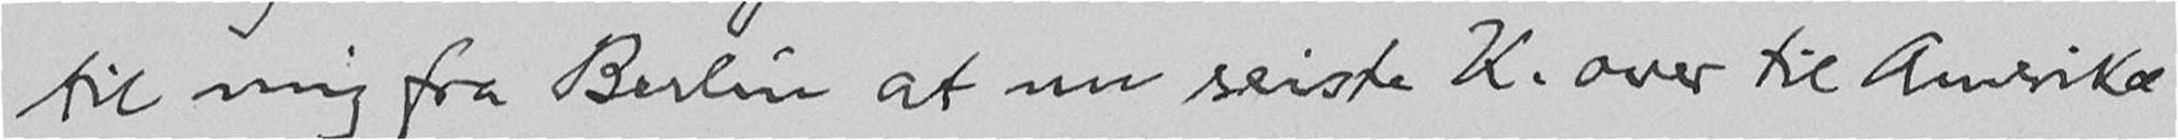til mig fra Berlin at nu reiste K. over til Amerika
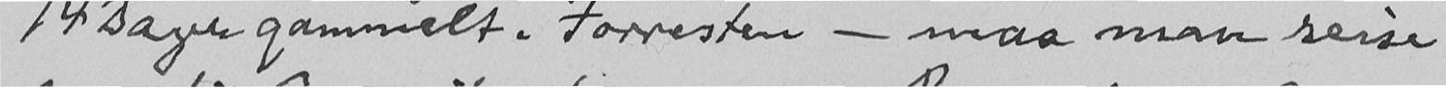14 Dager gammelt. Forresten - maa man reise
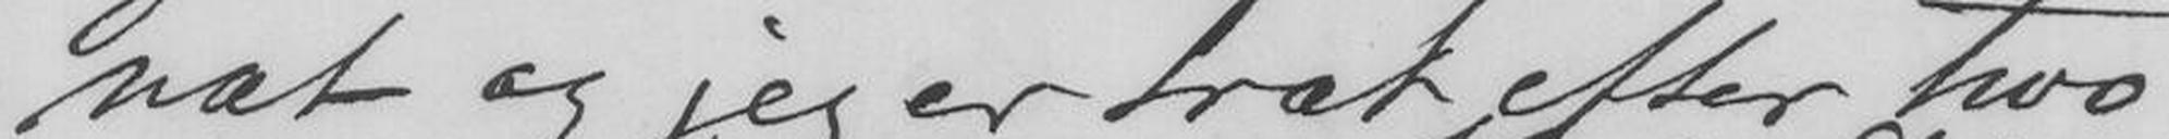nat og jeg er træt efter two
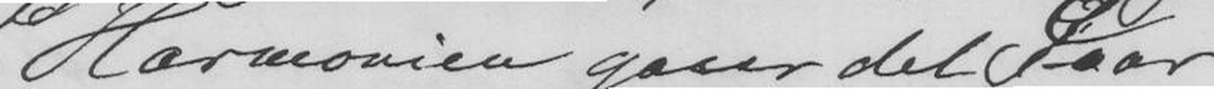Jo Harmonien gaaer det Iaar
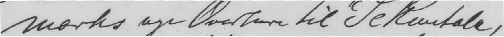mærks nye Overture til "Sekuntale,
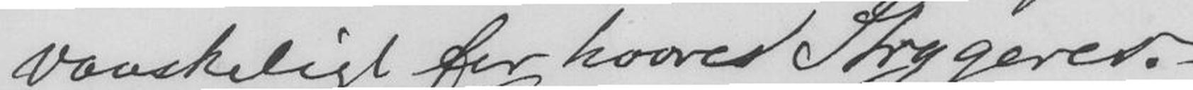vanskeligt for hvores Strygeres.
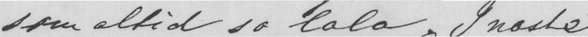som altid so lala. I næste
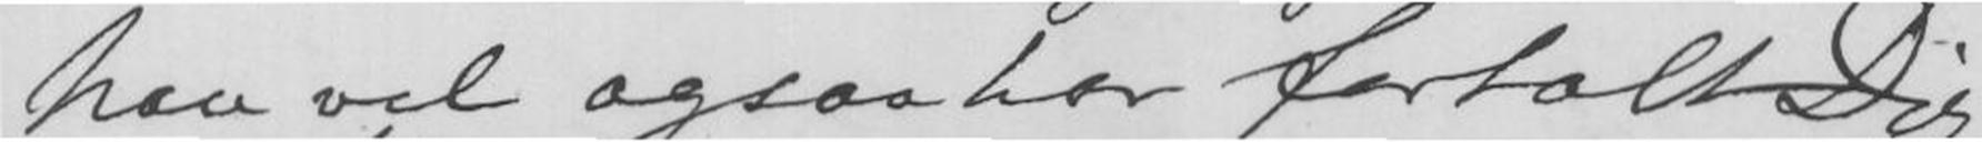han vel ogsaa har fortalt Dig
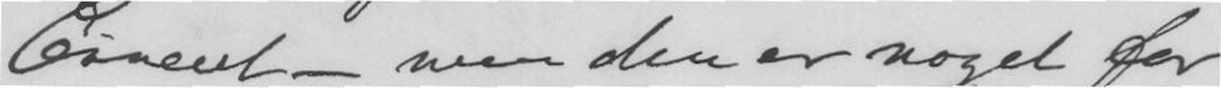Concert - men den er noget for
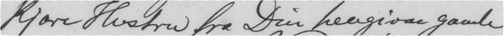kjære Hustru fra Din hengivne gamle
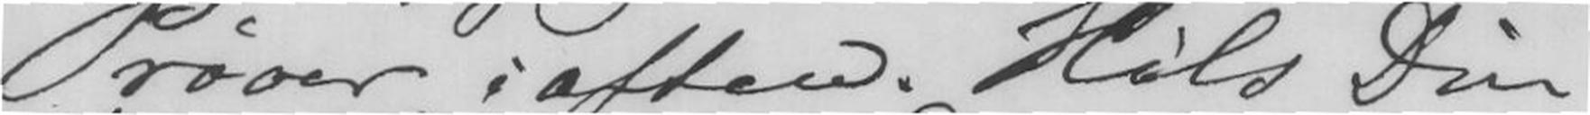Prøver i aften. Hils Din
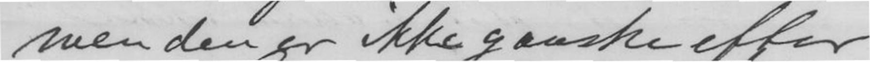men den er ikke ganske efter
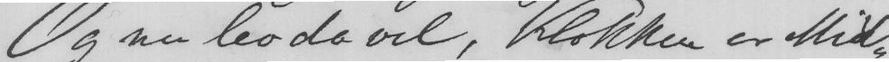Og nu lev da vel, Klokken er Mid-
